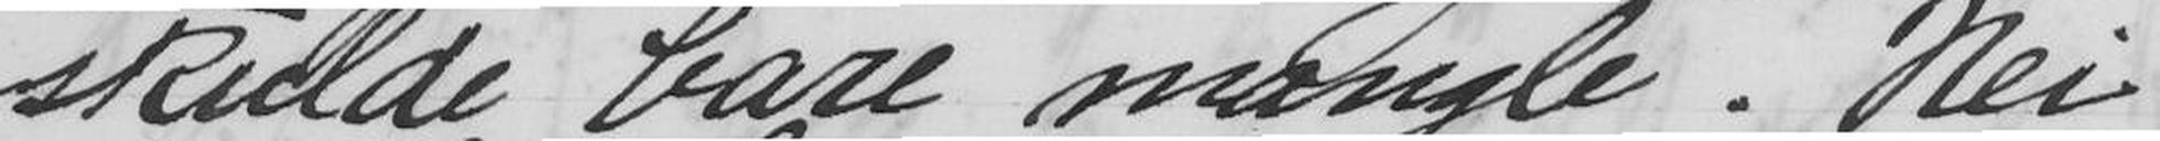skulde bare mangle. Nei,
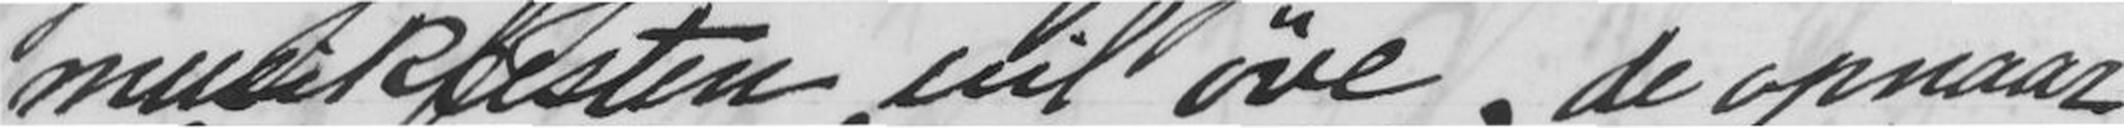musikfesten vil øve, de opnaar
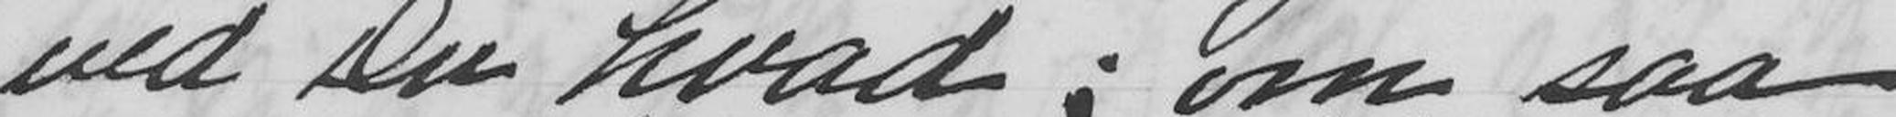ved Du hvad; om saa
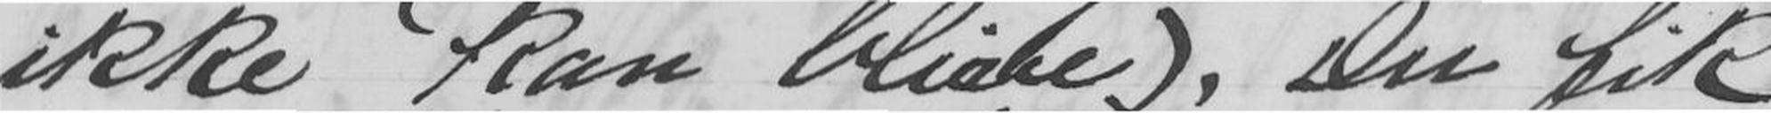ikke kan blive), Du fik
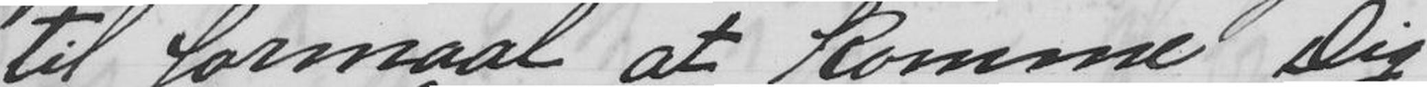til formaal at komme Dig
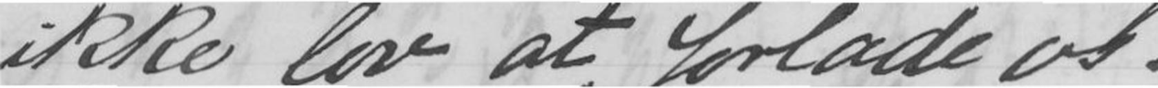ikke lov at forlade os.
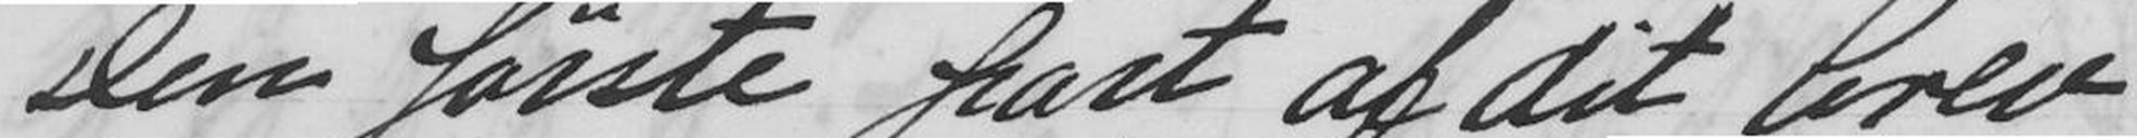Den første part af dit brev
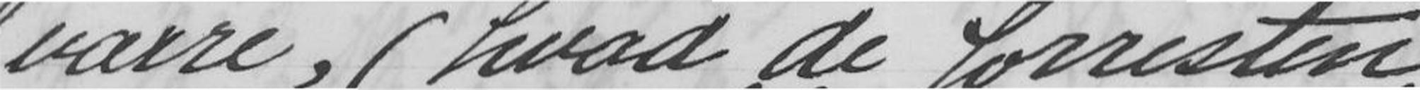værre, (hvad de forresten
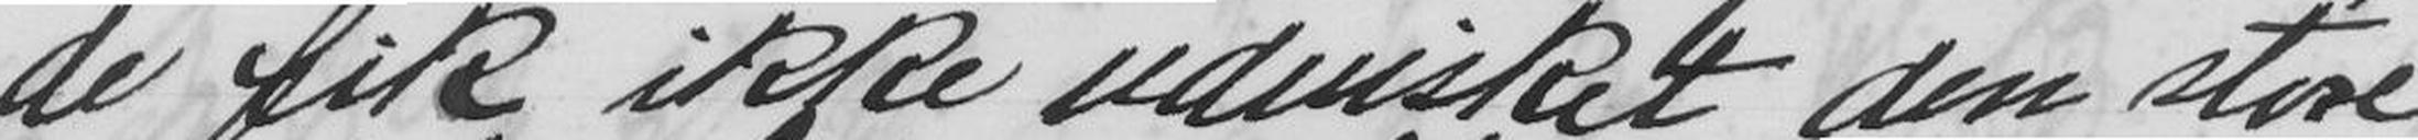de fik ikke udvisket den store
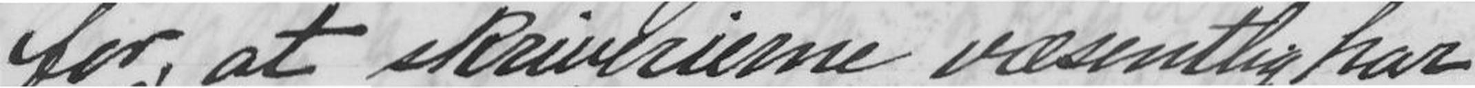for, at skriverierne væsentlig har
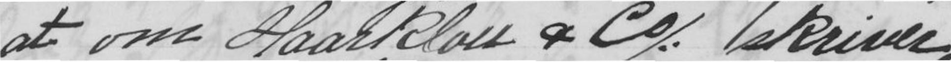at om Haarklou & Co/: skriver
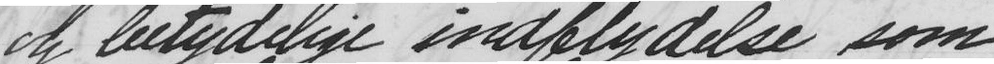og betydelige indflydelse som
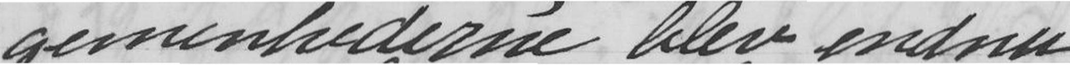gemenhederne blev endnu
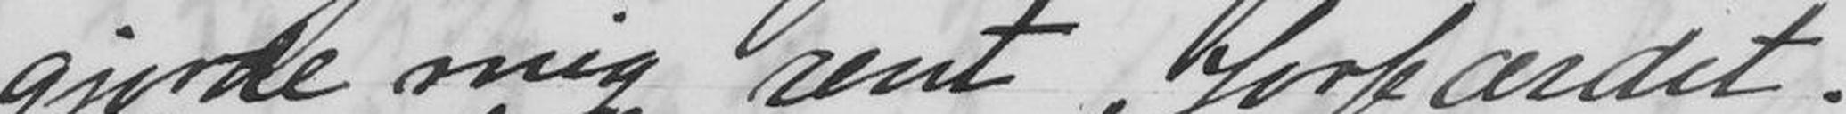gjorde mig rent forfærdet.
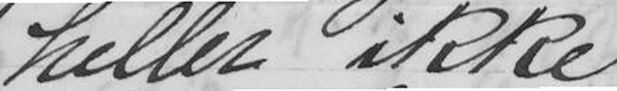heller ikke.
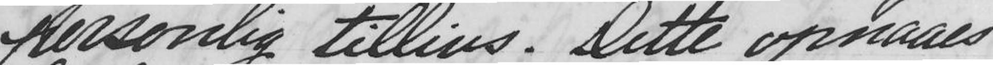personlig tillivs. Dette opnaas
